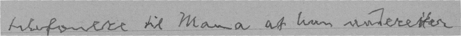telefonere til Mama at hun underetter
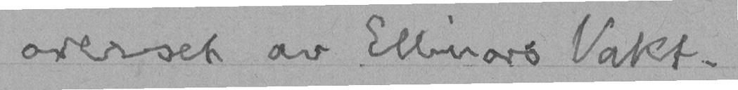overset av Ellinors Vakt.
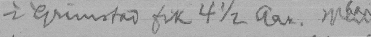i Grimstad fik 4½  Aar. Men
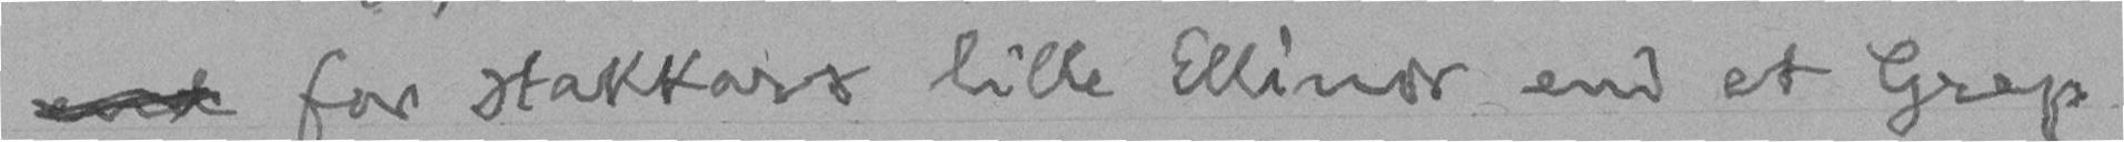end for stakkars lille Ellinor end et Grep
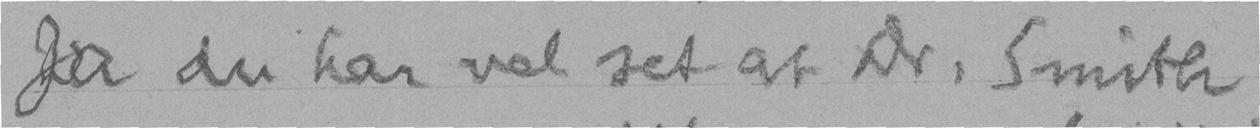Ja du har vel set at Dr. Smith
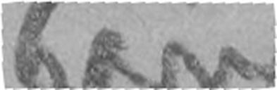han
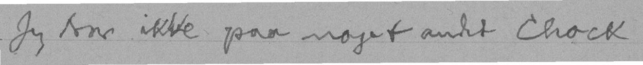Jeg tror ikke paa noget andet Chock
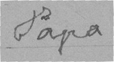Papa
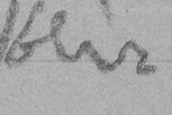blir
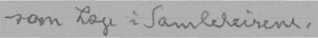som Læge i Samleleirene.
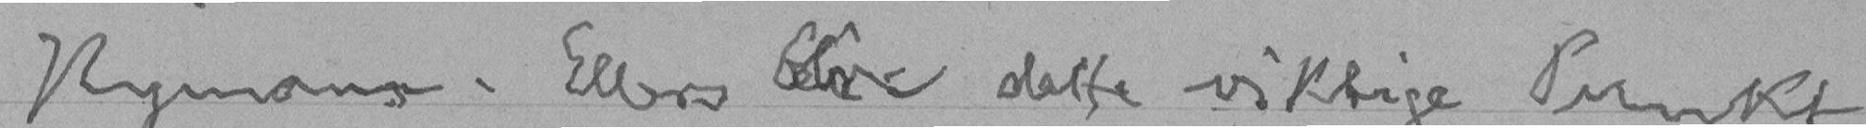Nymann. Ellers blir dette viktige Punkt
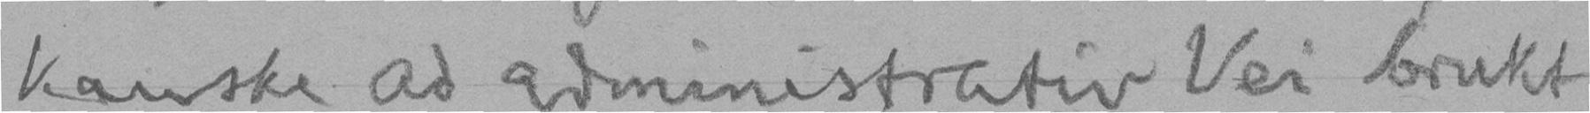kanske ad administrativ Vei brukt
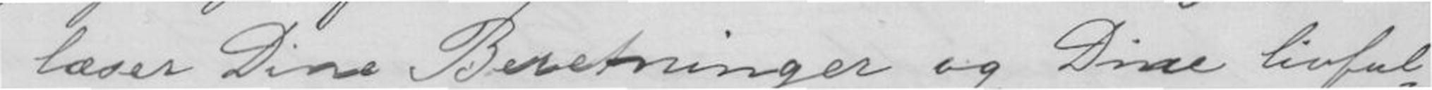læser Dine Beretninger og Dine livful-
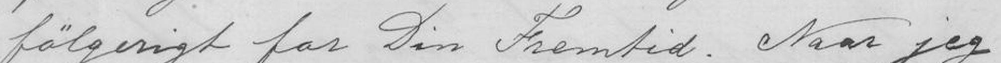følgerigt for Din Fremtid. Naar jeg
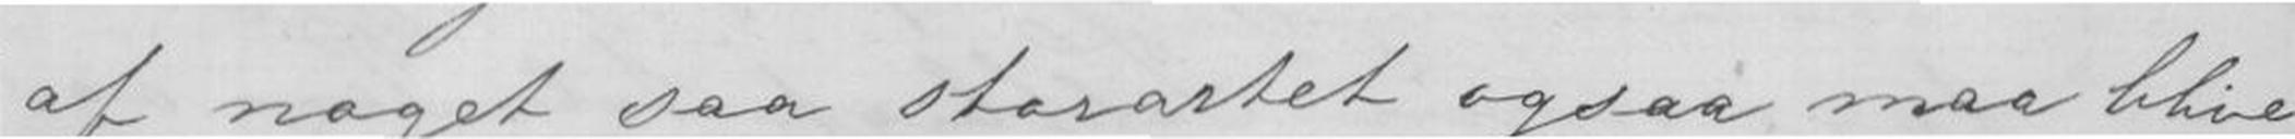af noget saa storartet ogsaa maa blive
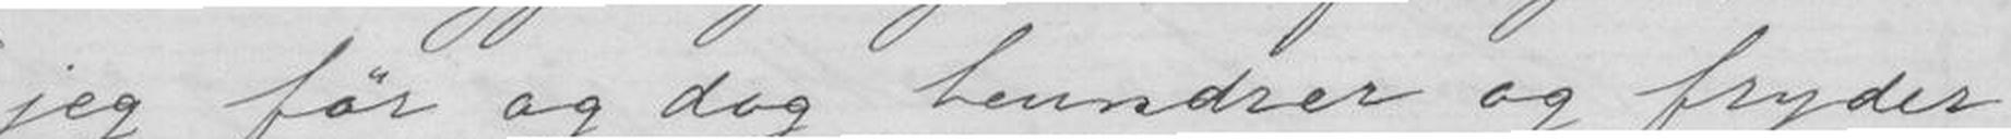jeg før og dog beundrer og fryder
